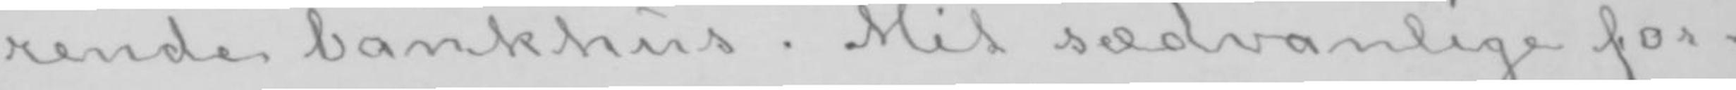rende bankhus. Mit sædvanlige for-
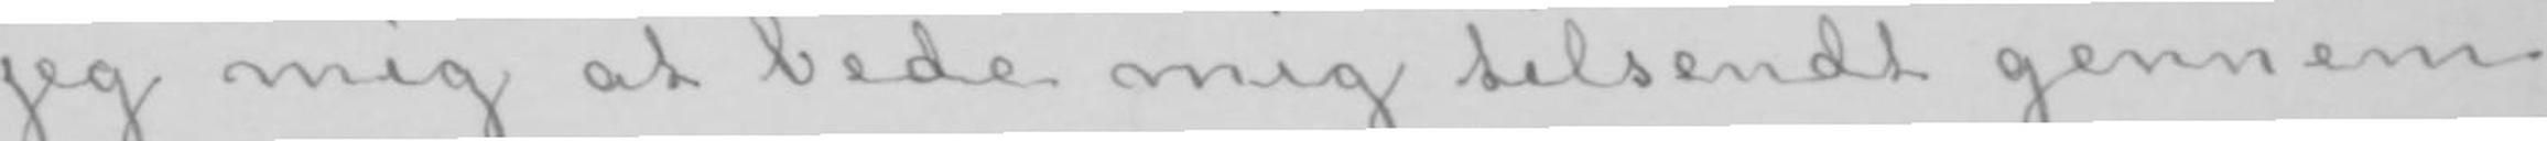jeg mig at bede mig tilsendt gennem
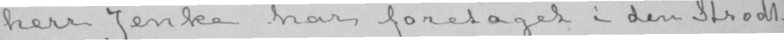herr Jenke har foretaget i den Strodt-
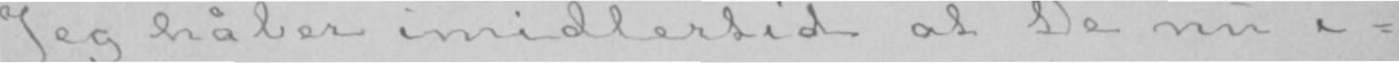Jeg håber imidlertid at De nu i-
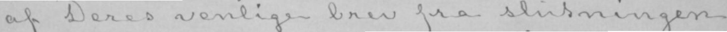af Deres venlige brev fra slutningen
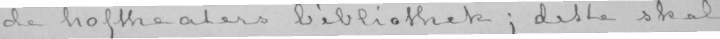de hoftheaters bibliothek; dette skal
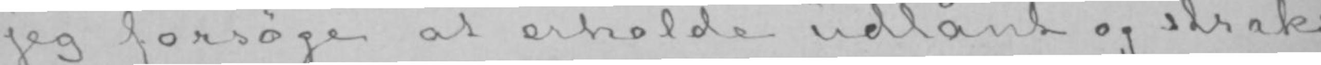jeg forsøge at erholde udlånt og straks
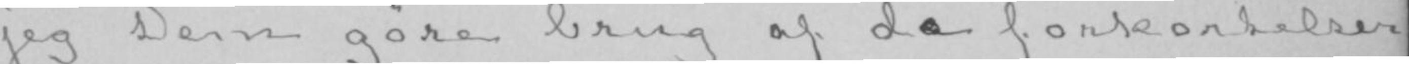jeg Dem gøre brug af de forkortelser
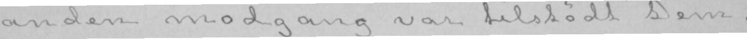anden modgang var tilstødt Dem.
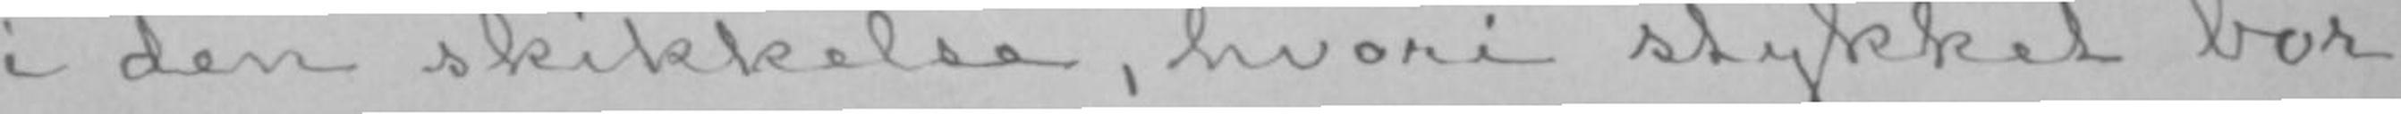i den skikkelse, hvori stykket bør
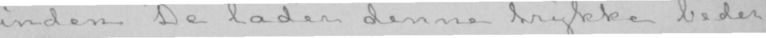inden De lader denne trykke beder
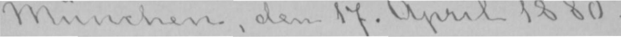München, den 17. April 1880.
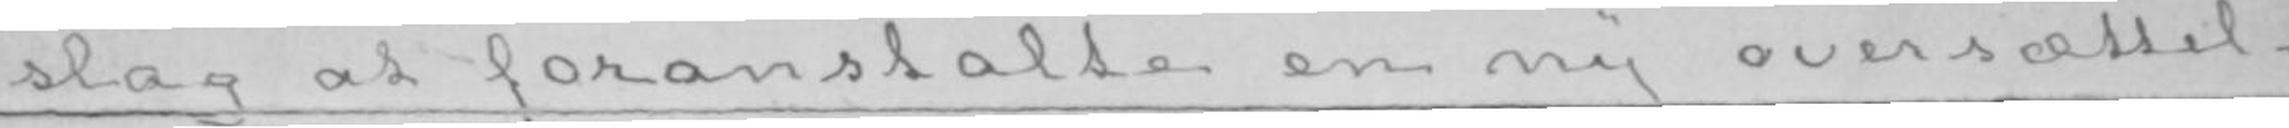slag at foranstalte en ny oversættel-
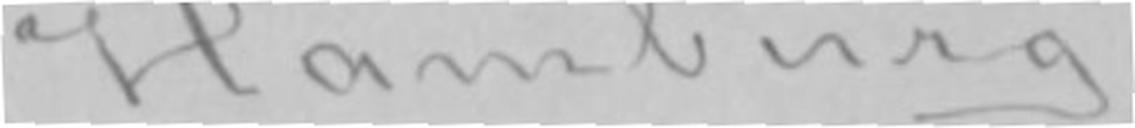Hamburg.
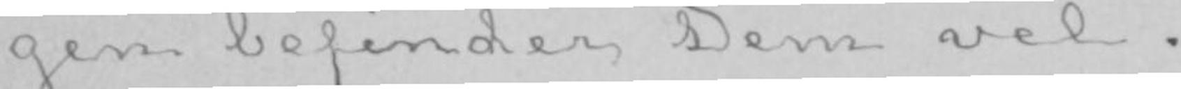gen befinder Dem vel.
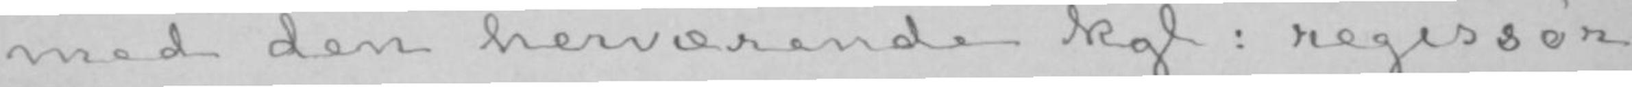med den herværende kgl: regissør
